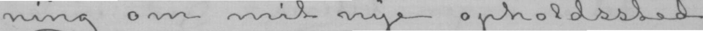ning om mit nye opholdssted,
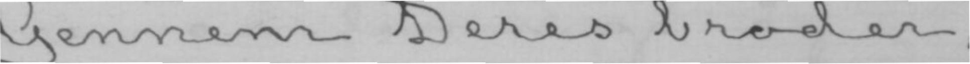Gennem Deres broder,
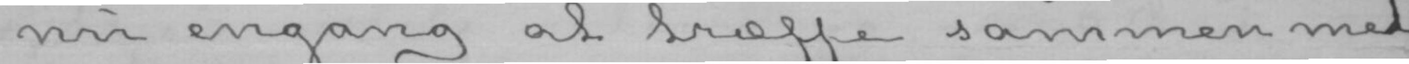nu engang at træffe sammen med
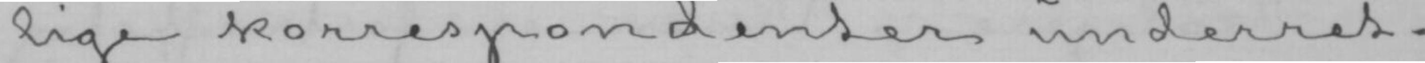lige korrespondenter underret-
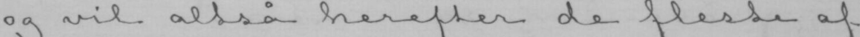og vil altså herefter de fleste af
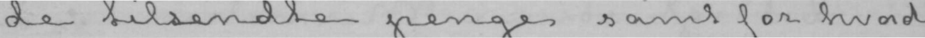de tilsendte penge samt for hvad
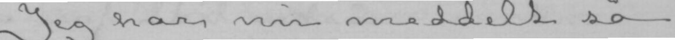Jeg har nu meddelt så
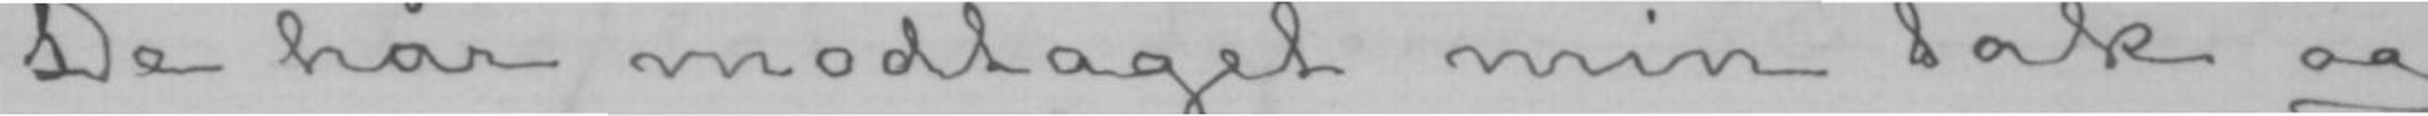De har modtaget min tak og
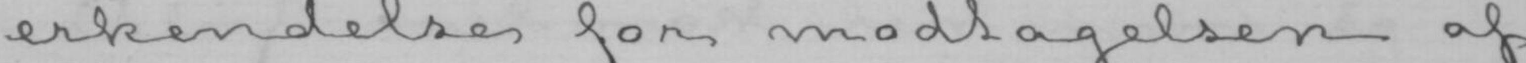erkendelse for modtagelsen af
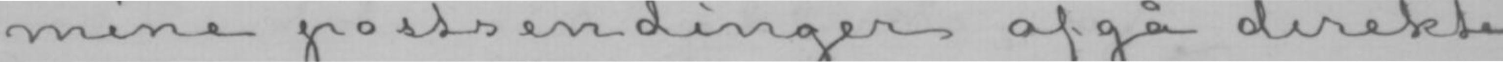mine postsendinger afgå direkte
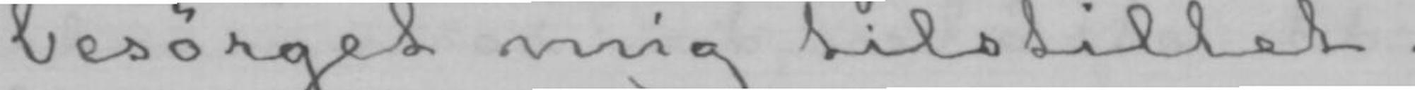besørget mig tilstillet.
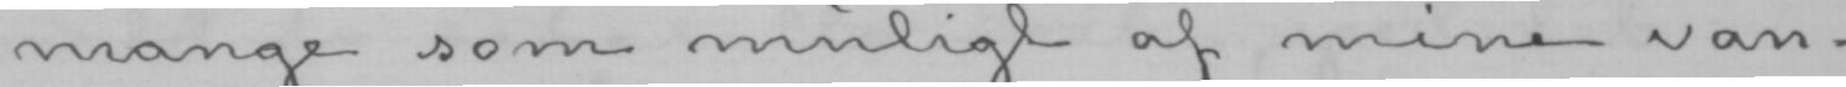mange som muligt af mine van-
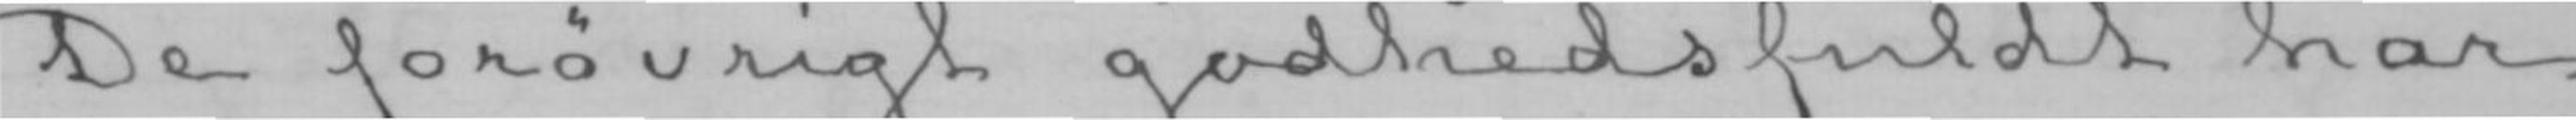De forøvrigt godhedsfuldt har
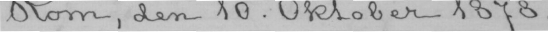Rom, den 10. Oktober 1878.
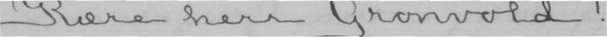Kære herr Grønvold!
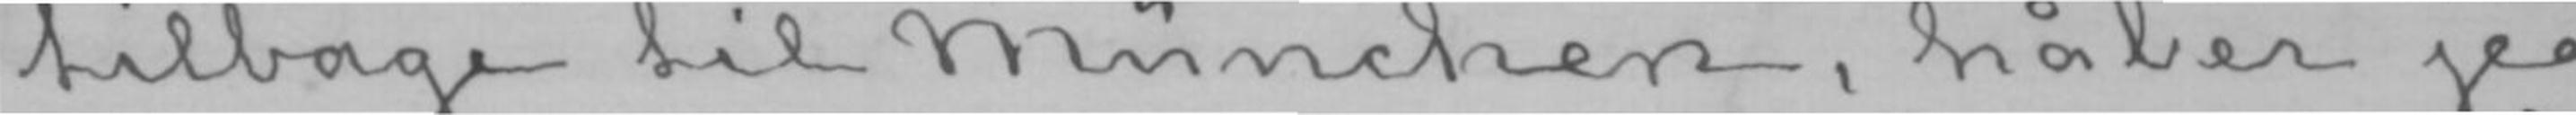tilbage til München, håber jeg
